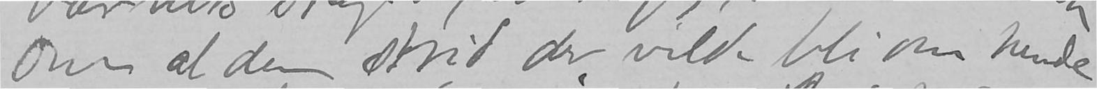om al den strid der vilde bli om hende
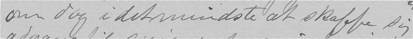om dog i detmindste at skafffe sig
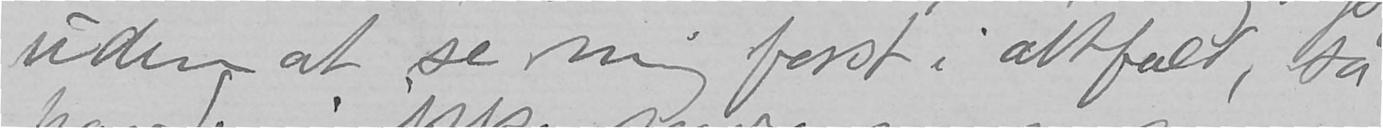uden at se mig først i altfald, så
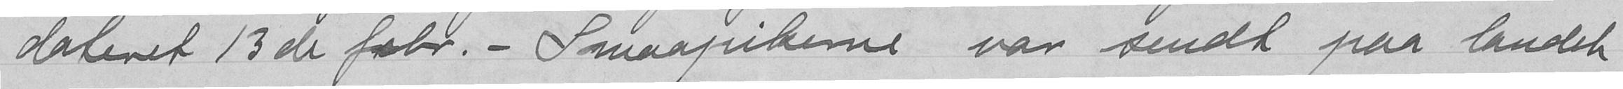dateret 13de febr. - Smaapikerne var sendt paa landet
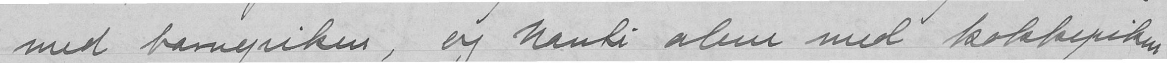med barnepiken, og Nanti alene med kokkepiken
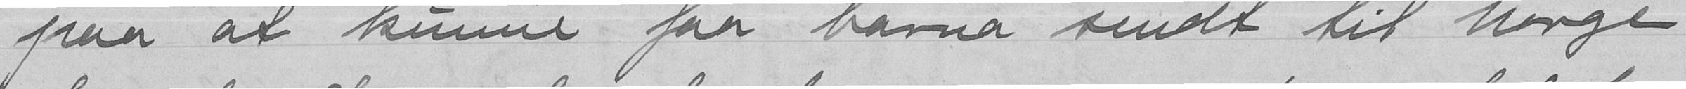paa at kunne faa barna sendt til Norge
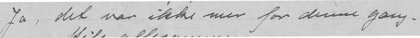Ja, det var ikke mer for denne gang.
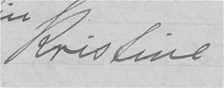Kristine
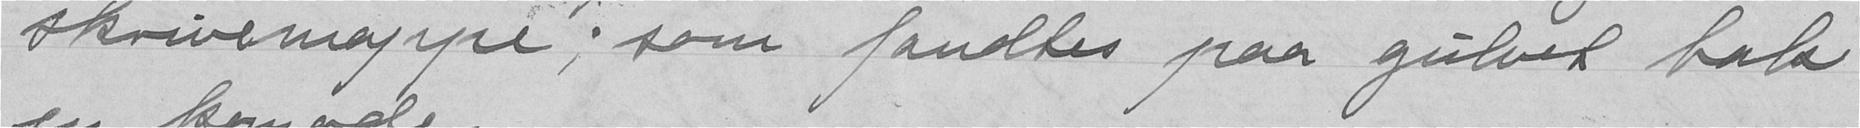skrivemappe, som fandtes paa gulvet bak
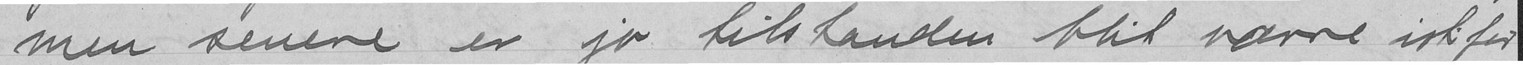men senere er jo tilstanden blit værre ist.for
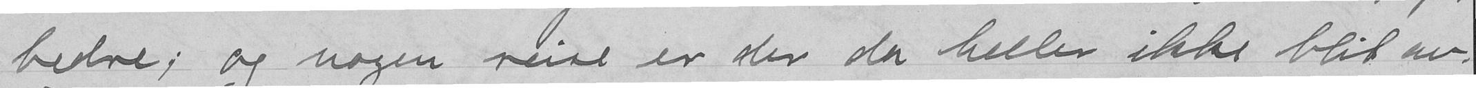bedre; og nogen reise er der da heller ikke blit av.
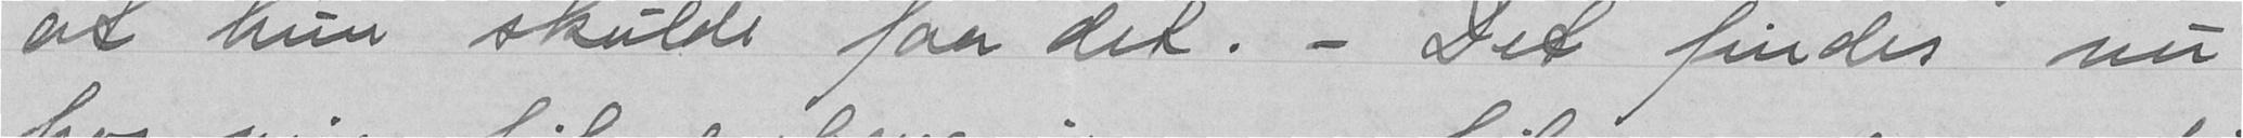at hun skulde faa det.- Det findes nu
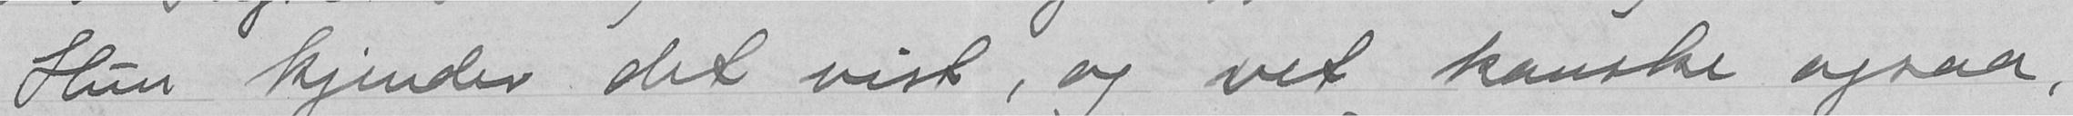Hun kjender det vist, og vet kanske ogsaa,
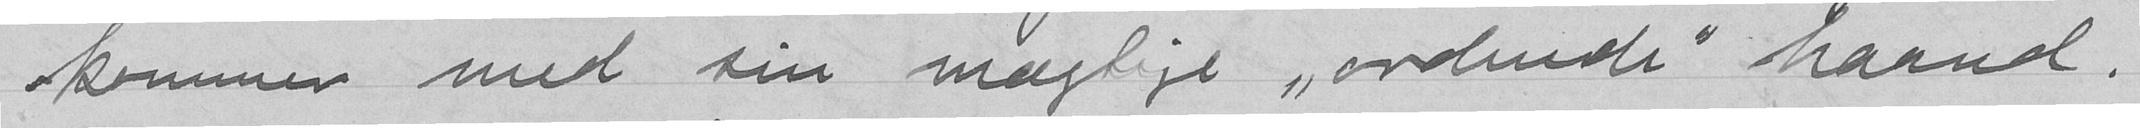kommer med sin magelige ordnede haand.
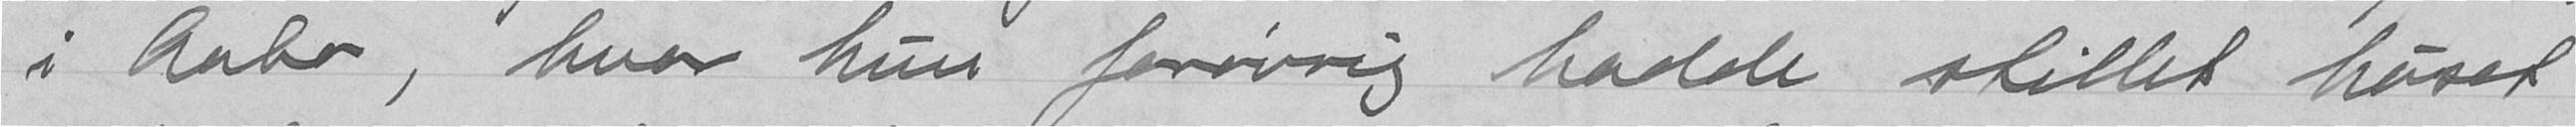i Aabo, hvor hun forøvrig hadde stillet huset
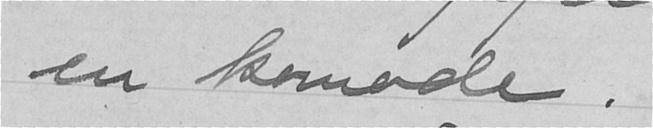en komode.
